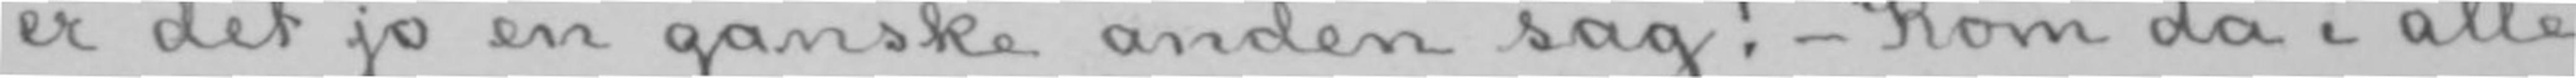er det jo en ganske anden sag!- Kom da i alle
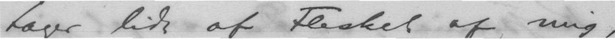tager lidt af Flesket af mig,
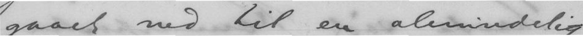gaaet ned til en almindelig
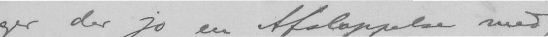ger der jo en Afslappelse med,
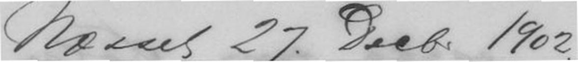Næsset 27. Decbr 1902.
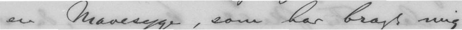en Mavesyge, som har bragt mig
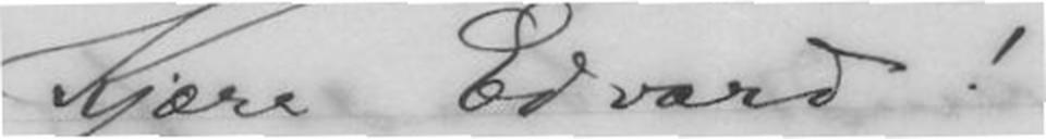Kjære Edvard!
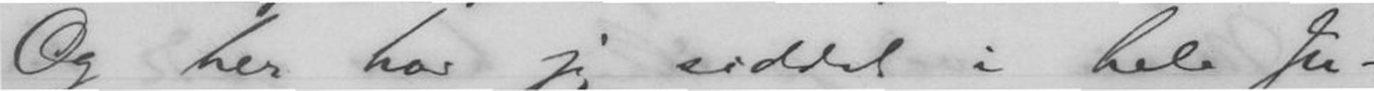Og her har jeg siddet i hele Ju-
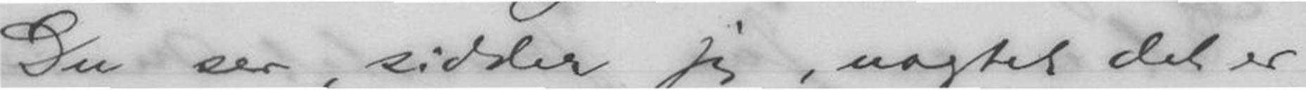Du ser, sidder jeg, uagtet det er
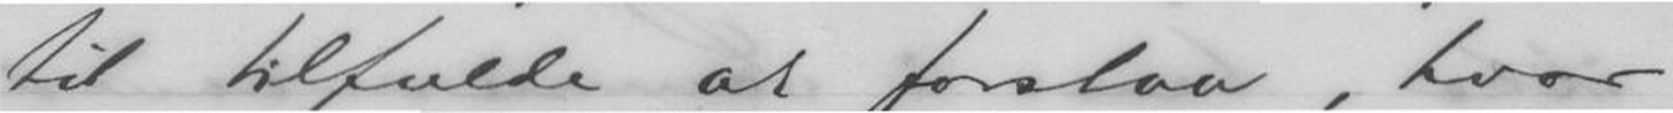til tilfulde at forstaa, hvor
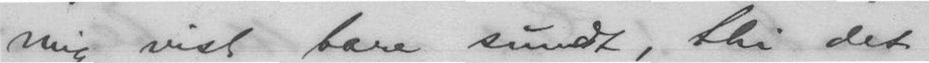mig vist bare sundt, thi det
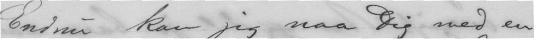Endnu kan jeg naa Dig med en
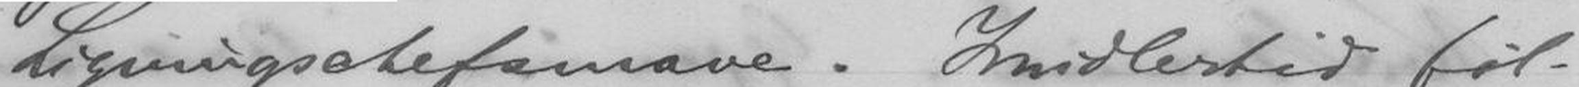Ligningschefmave. Imidlertid føl-
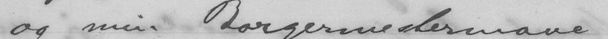og min Borgermestermave
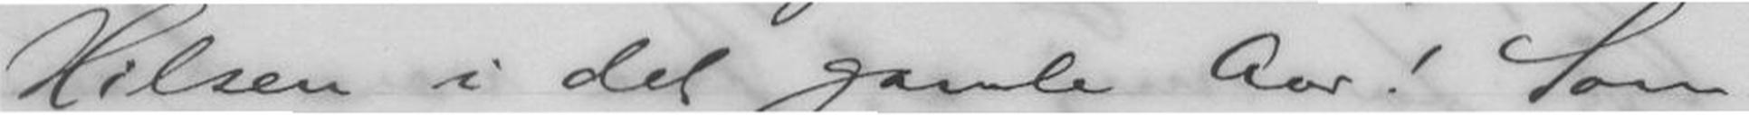Hilsen i det gamle Aar! Som
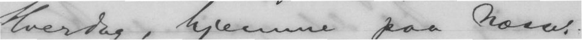Hverdag, hjemme paa Næsset.
